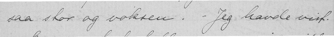saa stor og voksen. - Jeg havde vist
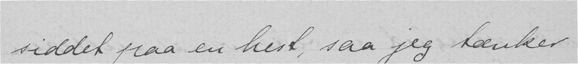siddet paa en hest, saa jeg tænker
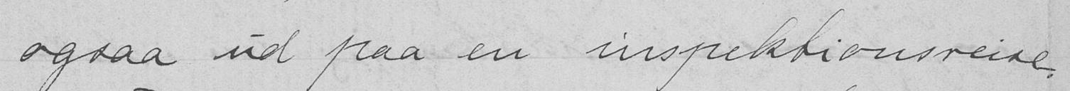ogsaa ud paa en inspektionsreise.
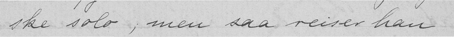ske solo, men saa reiser han
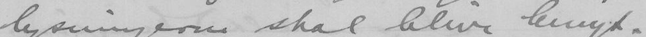lysningerne skal blive benyt-
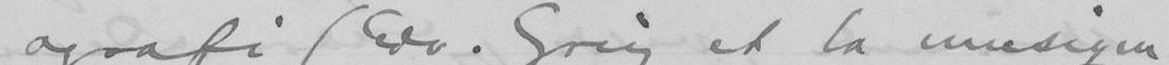ografi (Edv. Grieg et la musiqen
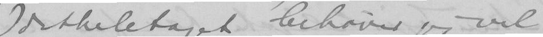Idetheletaget behøver jeg vel
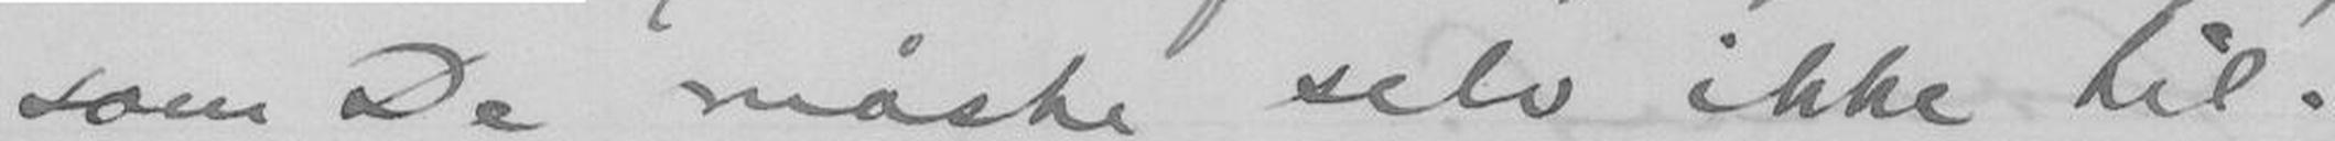som De måske selv ikke til-
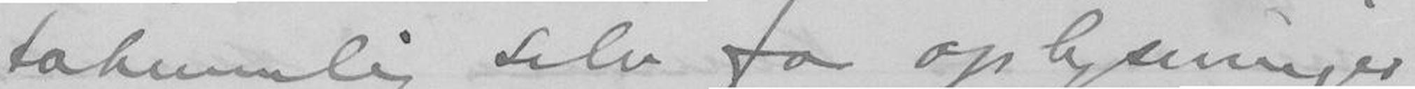taknemlig selv for oplysninger,
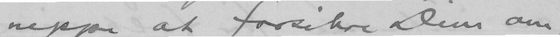neppe at forsikre Dem om,
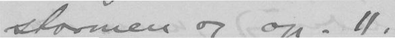stormen og op. 11.
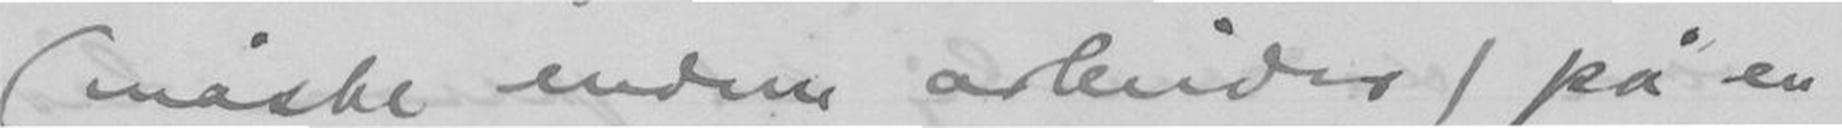(måske endnu arbeider) på en
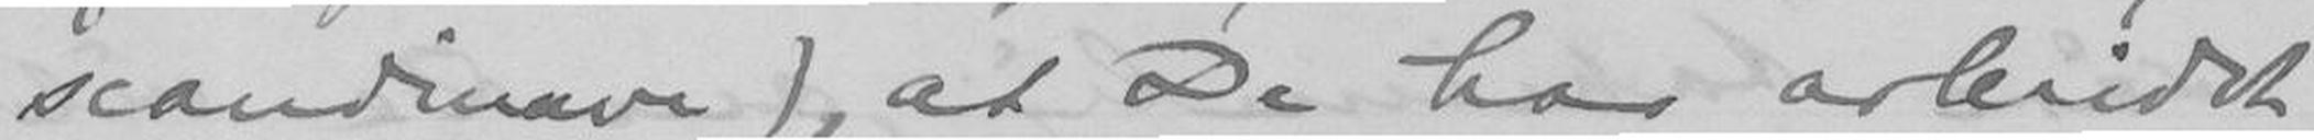scandinave), at De har arbeidet
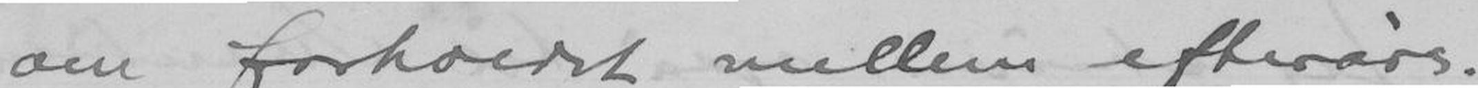om forhold mellem efterårs-
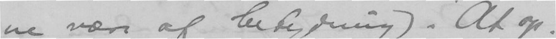ne være af betydning. At op-
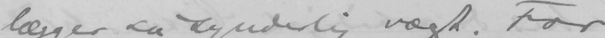lægge så synderlig vægt. For
